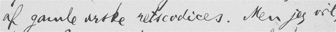af gamle irske retscodices. Men jeg vil,
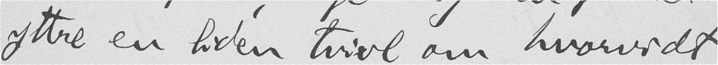yttre en liden tvivl om, hvorvidt
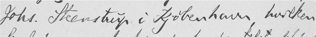Johs. Steenstrup i Kjøbenhavn, hvilken
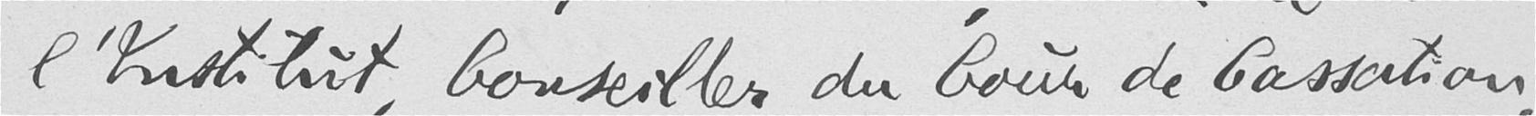l'Institut, Conseiller du Cour de Cassation,
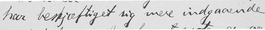har beskjæftiget sig mere indgaaende
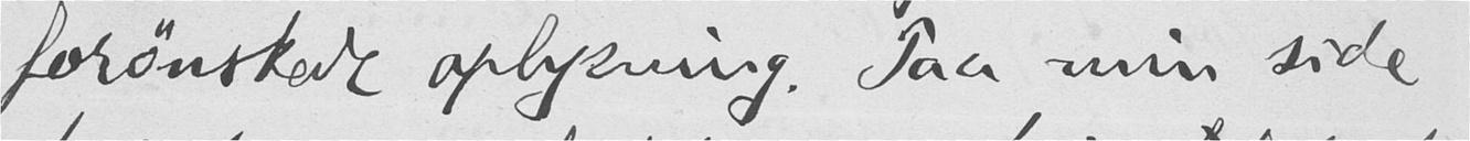forønskede oplysning. Paa min side
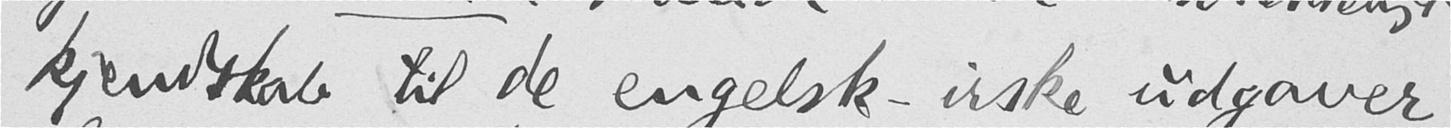kjendskab til de engelsk-irske udgaver
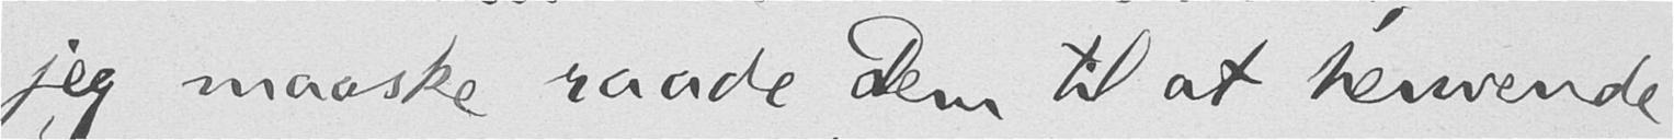jeg maaske raade dem til at henvende
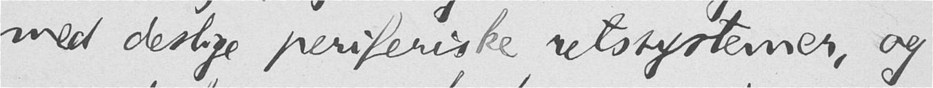med deslige periferiske retssystemer, og
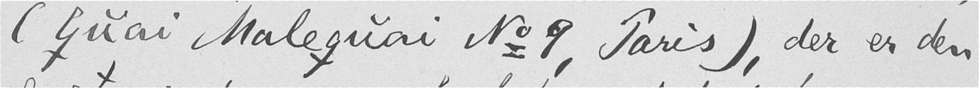(Quai Malequai No 9, Paris), der er den
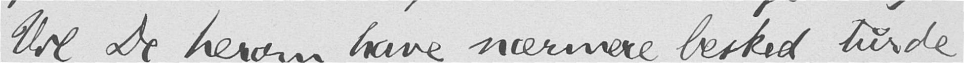Vil De herom have nærmere besked, turde
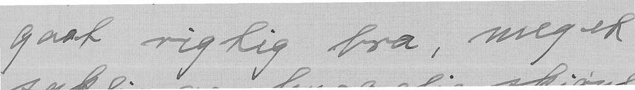gaat rigtig bra, meget
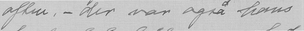aften, - der var også hans
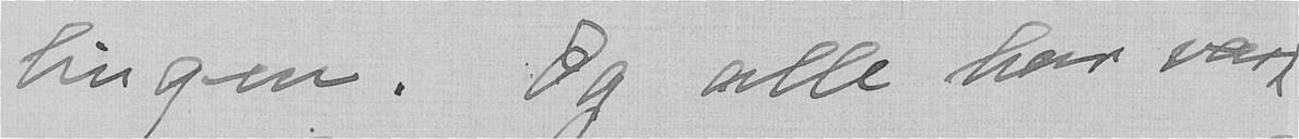lingen. Og alle har vært
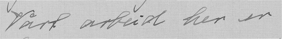Vårt arbeid her er
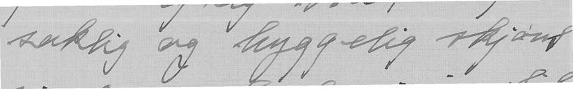saklig og hyggelig skjønt
